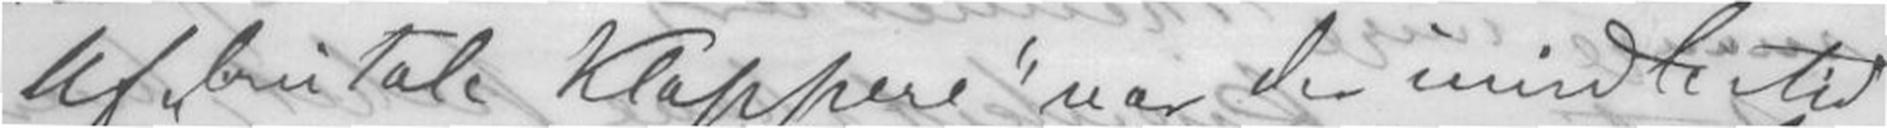Af brutale Klappere var der imidlertid
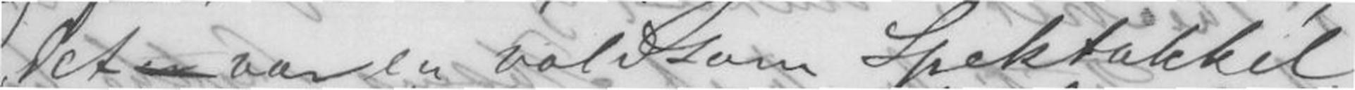det er var en voldsom Spetakkel;
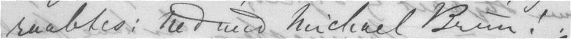raabtes: ned med Michael Brun!
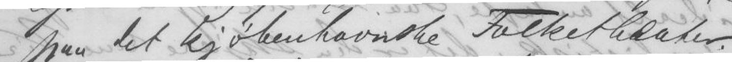paa det kjøbenhavnske Folketheater;
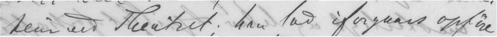teur ved Theatret; han lad iforgaars opføre
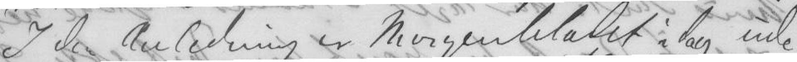I den Anledning er Morgenbladet i dag ude
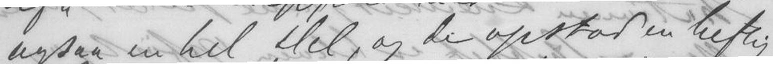ogsaa enhel Del, og de opstod en heftig
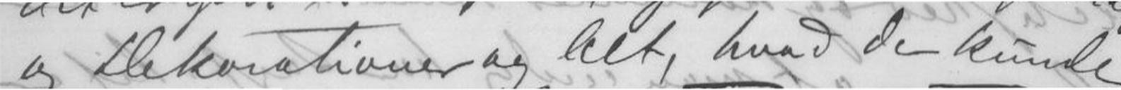og Dekorationer og Alt, hvad der kunde
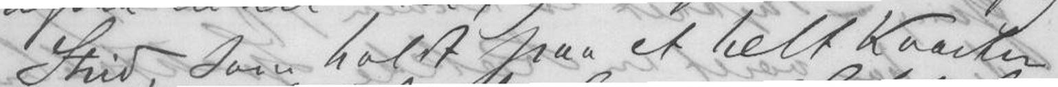Strid, som holdt paa et helt Kvarter
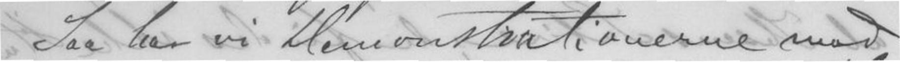Saa har vi Demonstrationerne mod
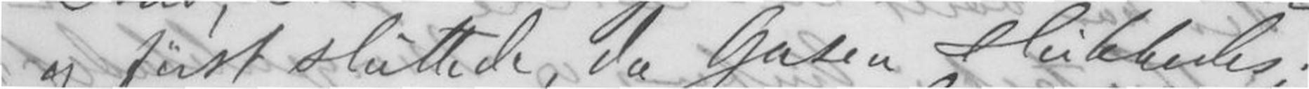og først sluttede, da Gasen slukkedes;
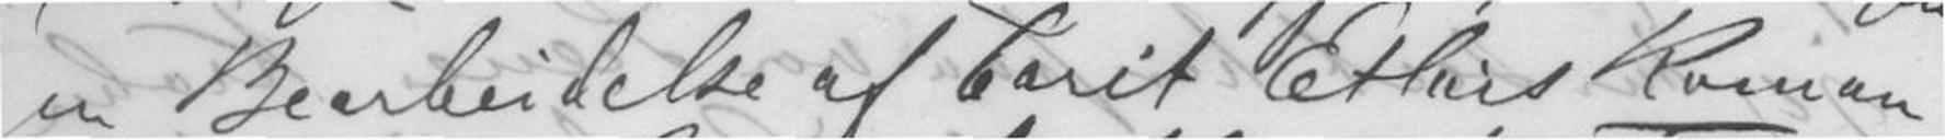en Bearbeidelse af Carit Etlars Roman
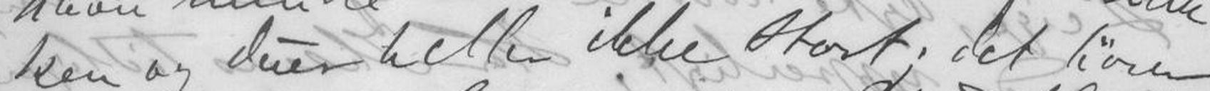ken og duer heller ikke stort, det hører
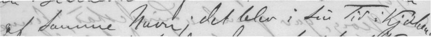af samme Navn; det blev i sin Tid i Kjøben-
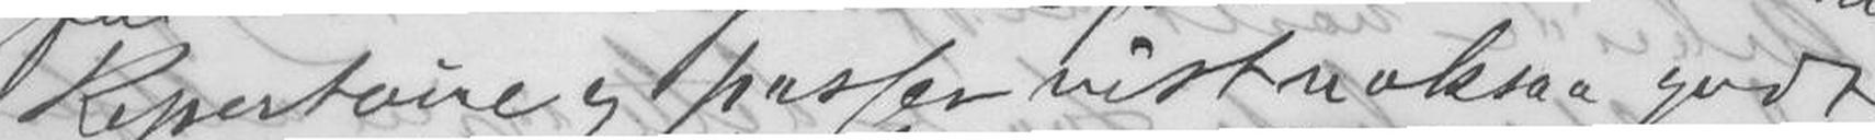Repertoire og passer vistnok godt
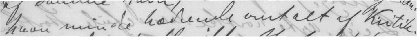havn mindre hædrende omtalt af Kritik-
